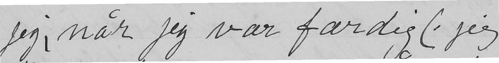jeg, når jeg var færdig (jeg
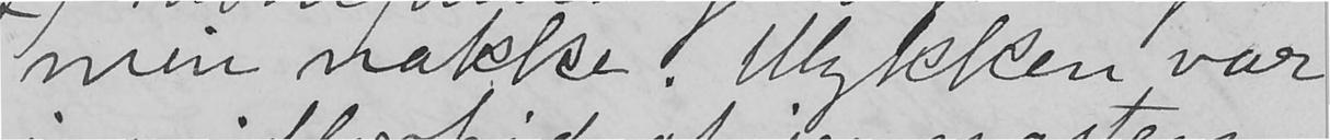min nakke. Ulykken var
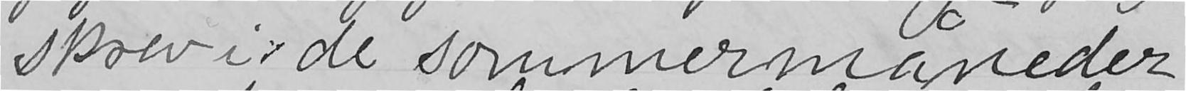skrev i de sommermåneder
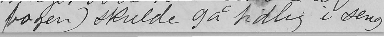bogen) skulde gå tidlig i seng
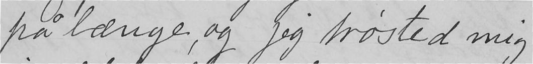på længe, og jeg trøsted mig
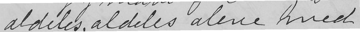aldeles, aldeles alene hved
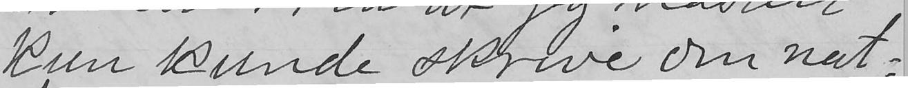kun kunde skrive om nat-
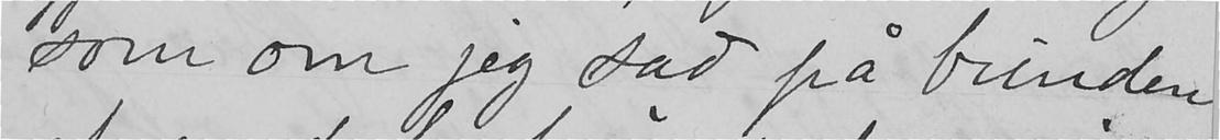som om jeg sad på bunden
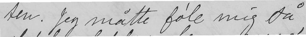ten. Jeg måtte føle mig så
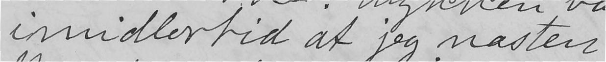imidlertid at jeg næsten
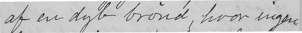af en dyb brønd, hvor ingen
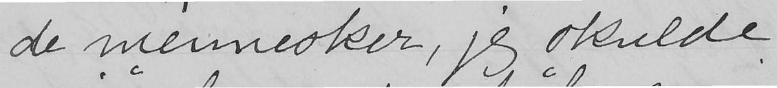de mennesker, jeg skulde
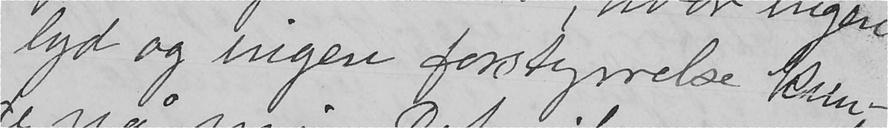lyd og ingen forstyrrelse kun-
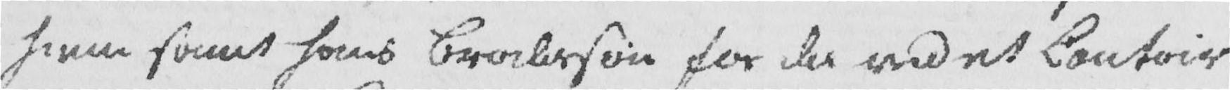hiem samt hans Brodersøn for der ved et Contoir
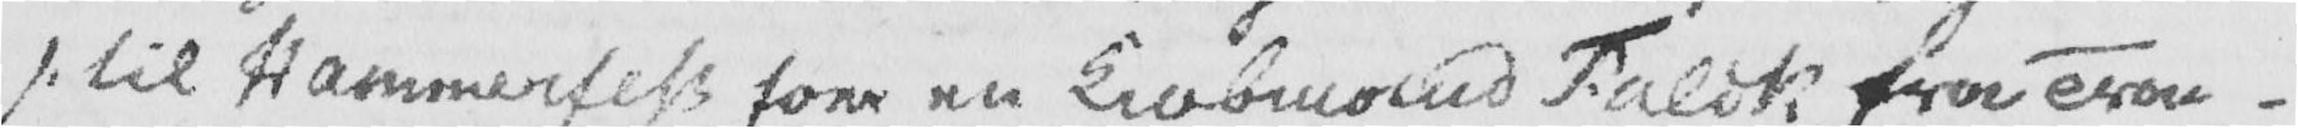/: til Hammerfest foer en Kiøbmand Falck fra Tron-
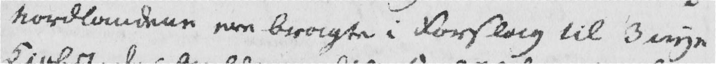Nordlandene ere bragte i Forslag til 3 nye
